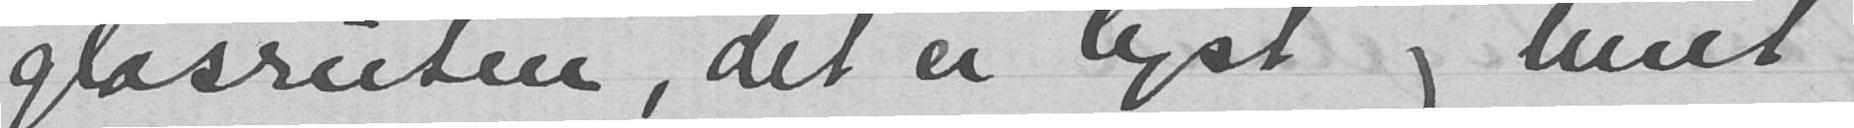glasruten, det er lyst og lunt
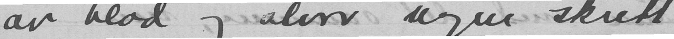av blod og alvor nogen skritt
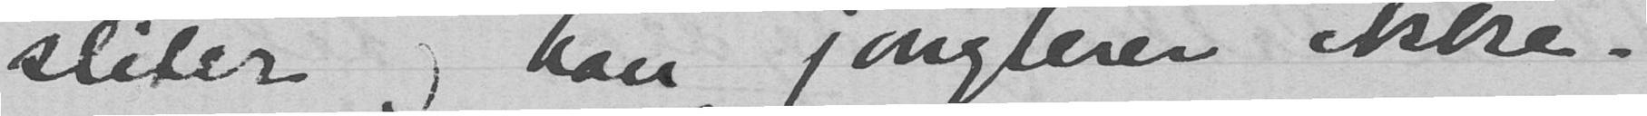sliter og han jonglerer ikke.
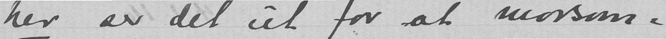her ser det ut for at morsom-
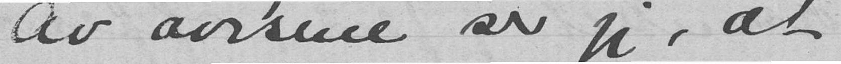Av avisene ser jeg, at
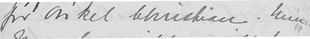for onkel Christian. Men
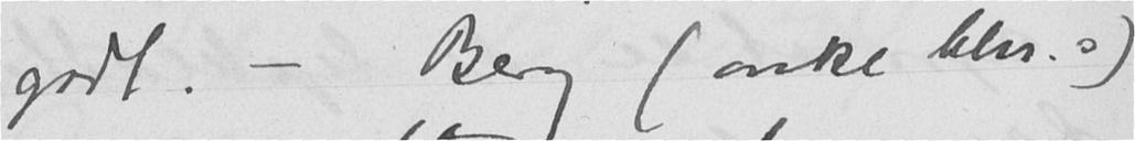godt. - Berg (onkel Chr.s)
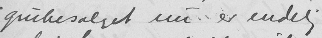grubesalget nu er endelig
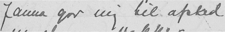Janna gav mig til afsked
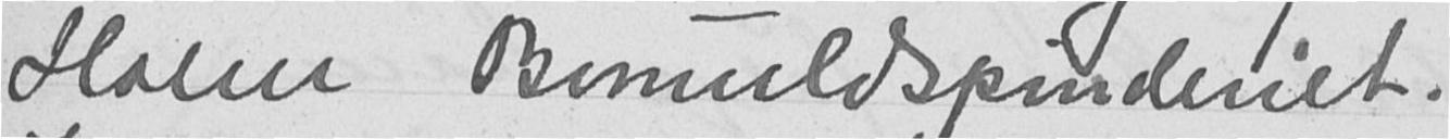Holm Bomuldspinderiet.
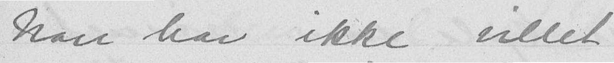han har ikke villet
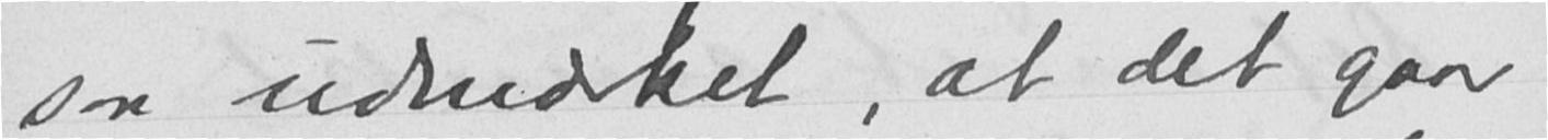saa udmerket, at det gaar
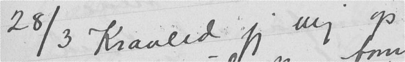28/3 Kravled jeg mig op
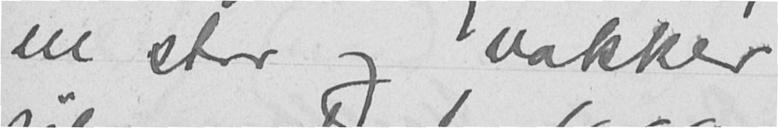en stor og vakker
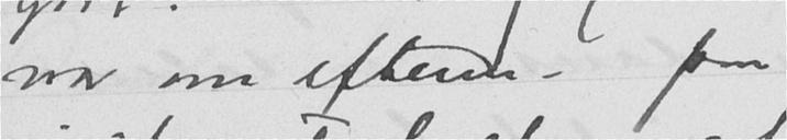var om efterm. paa
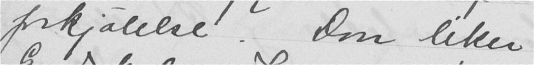forkjølelse. Don liker
